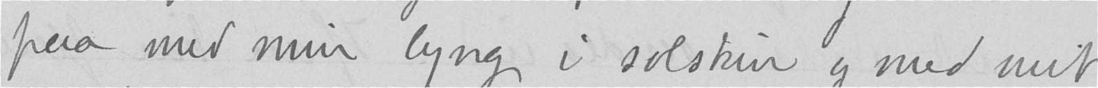paa med min lyng i solskin og med mit
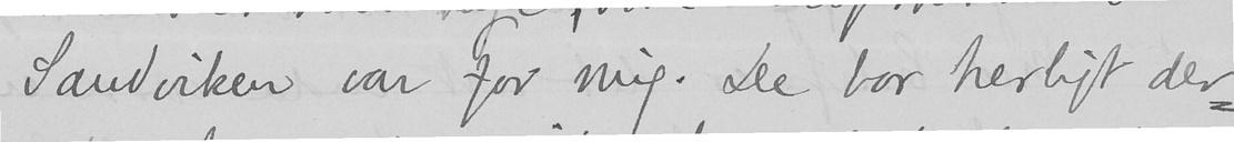Sandviken var for mig. De bor herligt der
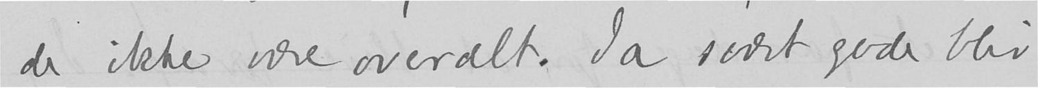de ikke være overalt. Ja svært gode blir
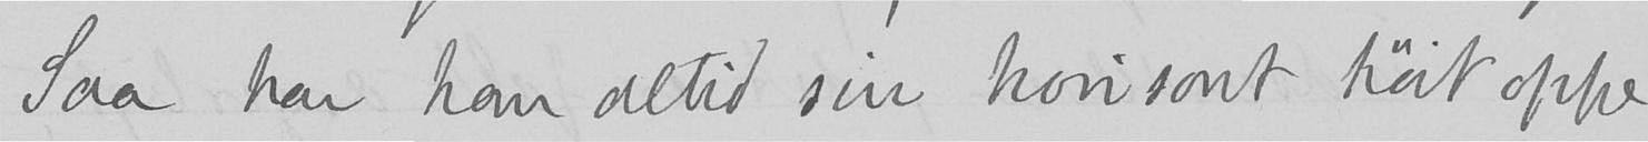Saa har han altid sin horisont høit oppe
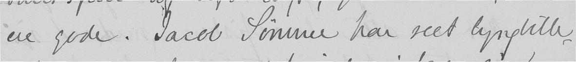ere gode. Jacob Sømme har seet lyngbille-
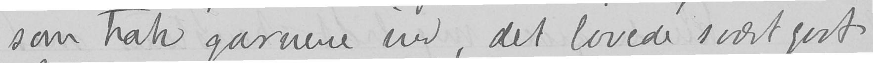som trak garnene ind, det lovede svært godt
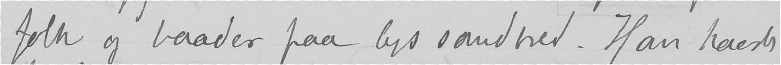folk og baader paa lys sandhed. Han havde
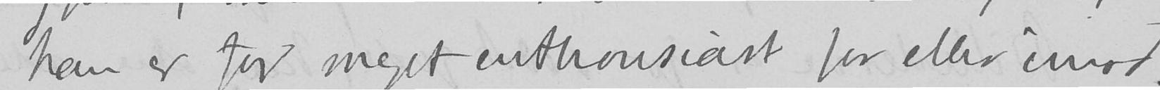han er for meget enthousiast for eller imod.
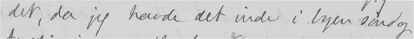det, da jeg havde det inde i byen søndag
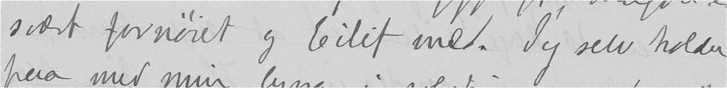svært fornøiet og Eilif med. Jeg selv holder
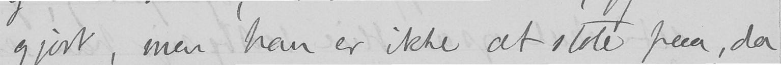gjort, men han er ikke at stole paa, da
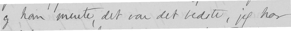og han mente, det var det bedste, jeg har
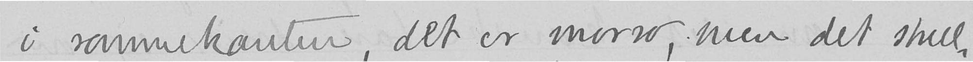i rammekanten, det er morro, men det skuld-
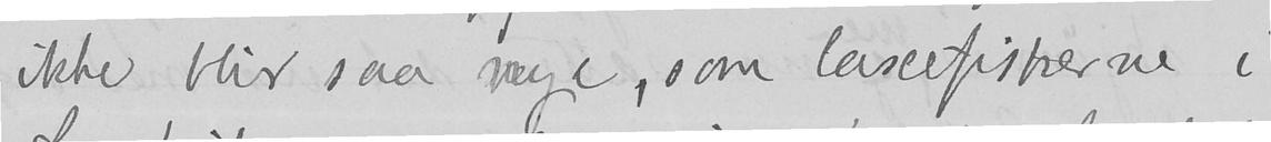ikke blir saa nye, som laxefiskerne i
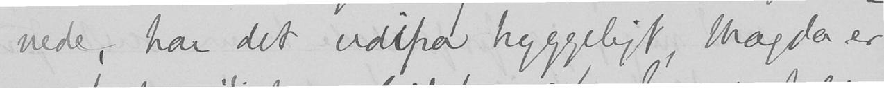nede, har det udifra hyggeligt, Magda er
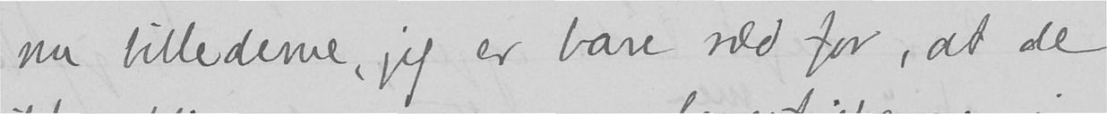nu billederne, jeg er bare ræd for, at de
